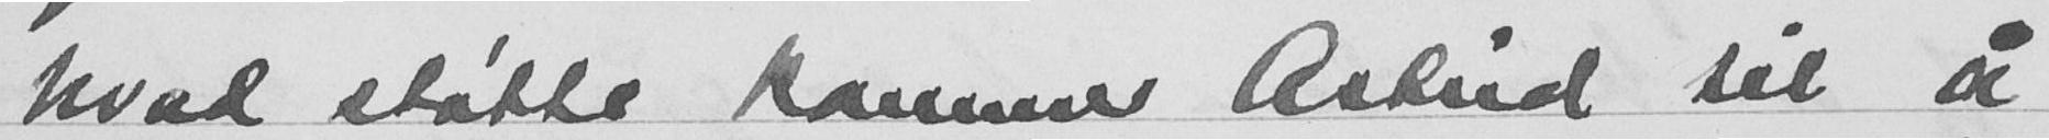hvad støtte kommer Astrid til å
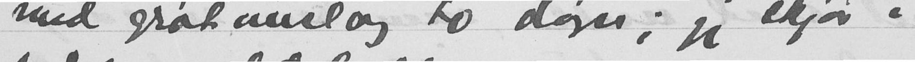med grøt omlag to døgn; jeg skjør i
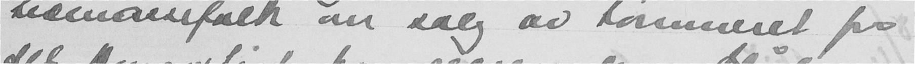tremassefolk om salg av tømmeret for
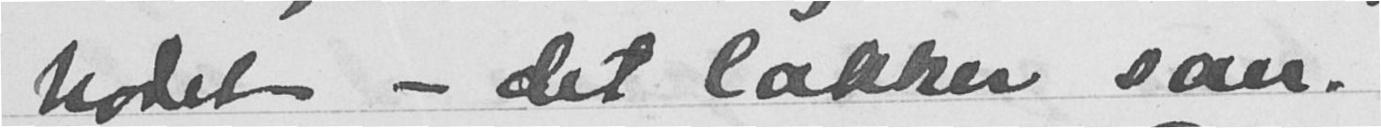hodet - det takker oss.
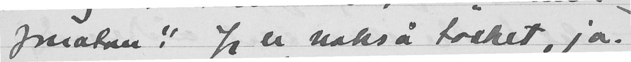Jonatan". Jeg er nokså tosket, ja.
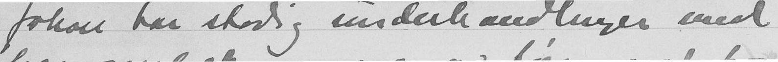Johan har stadig underhandlinger med
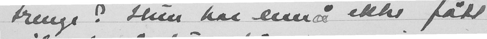trenge? Hun har ennå ikke fått
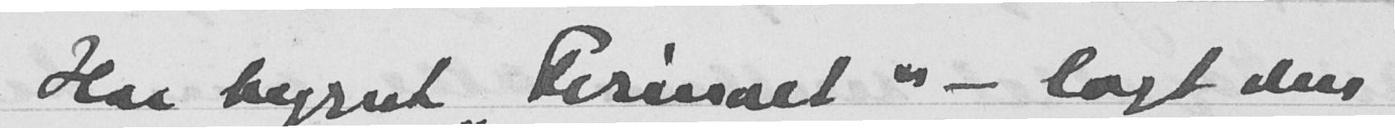Har begynt "Firmaet" - lagt den
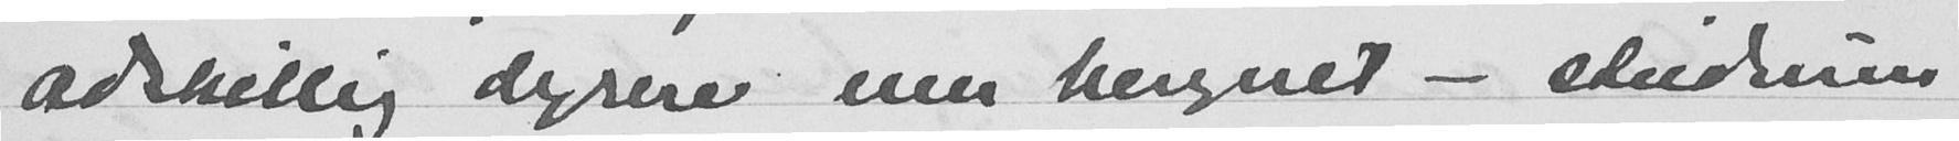adskillig dyrere end beregnet - studium
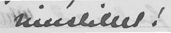innstillet!
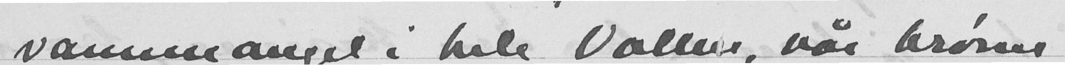varmemangel i hele Vollen, vår butikk
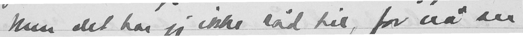Men det har jeg ikke råd til for nå ser
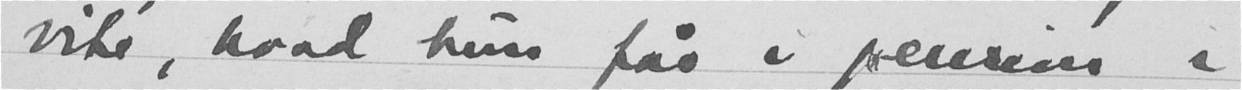vite, hvad hun får i pension i
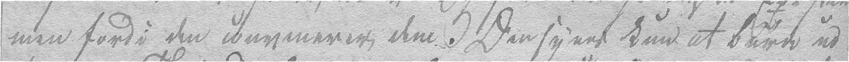men fordi den bavinerer dem. Den synes kun at burde ud-
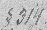§ 314.
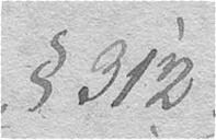§ 312.
